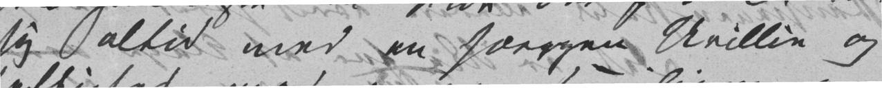sig altid med en særegen Uvillie og
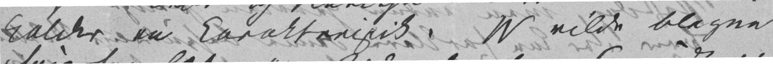kaldes en Carakteristik. W vilde blegne
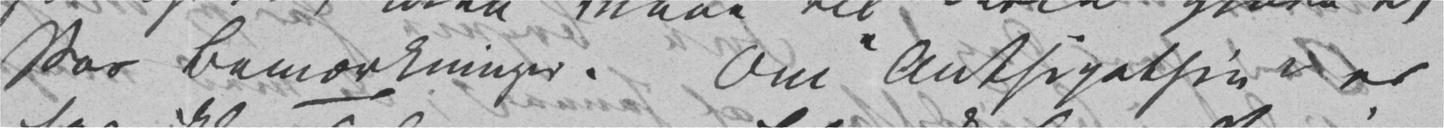Par Bemærkninger. Om «Anthipathie» er
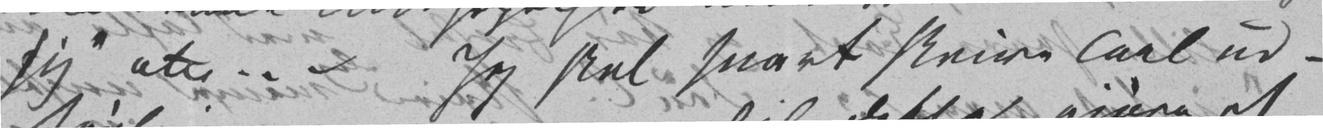sig» etc. – – Jeg skal snart skrive Carl ud-
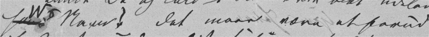hans W.s Navn? Det maae være et forud-
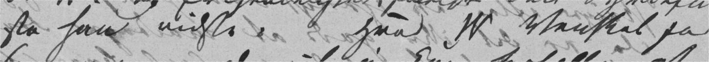-ste han vidste. Hvad W Venskab for
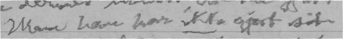Man han har ikke gjort sit.
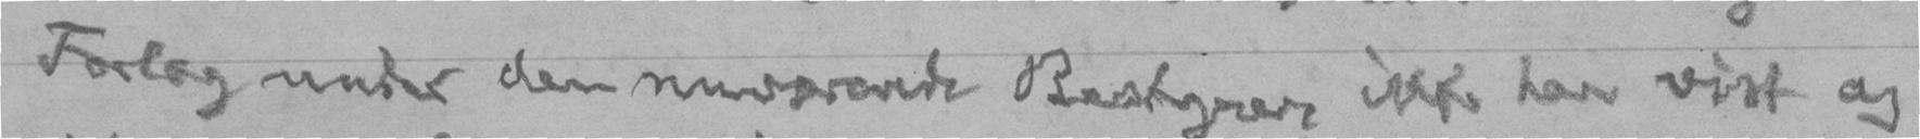Forlag under den nuværende Bestyrer ikke har vist og
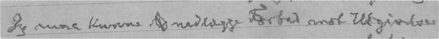Jeg maa kunne  nedlægge Forbud mot Utgivelse
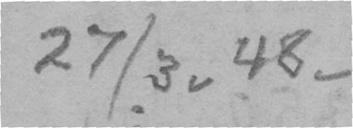27/3. 48.
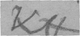KH
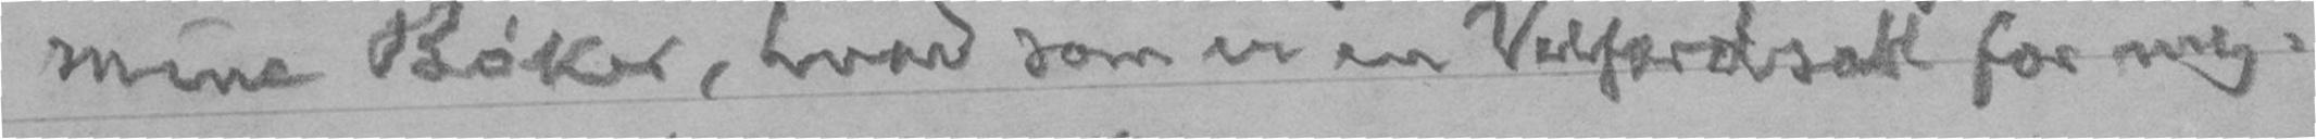mine Bøker, hvad som er en Velfærdsak for mig.
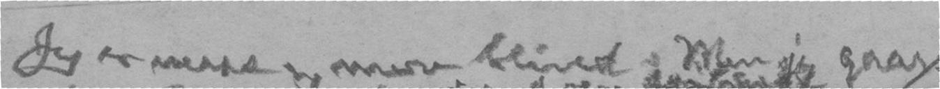Jeg er mere og mere blind. Men jeg gaar
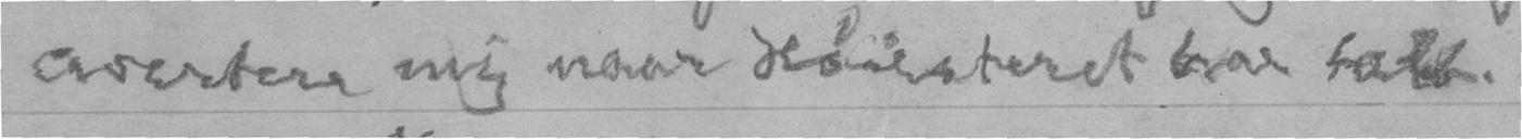avertere mig naar Høiesteret har talt.
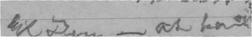til Dem - at han
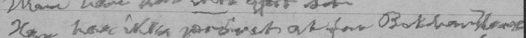Han har ikke prøvet, at faa Bokhandlerne
